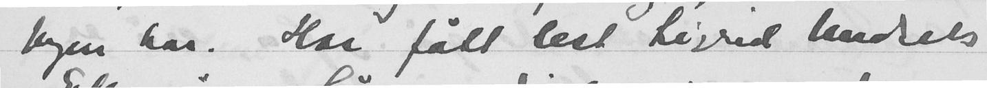byen har. Har fått lest Sigrid Undsets
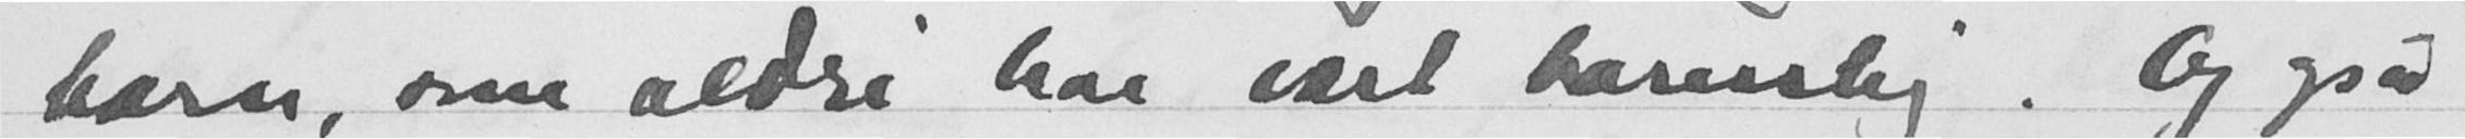barn, som aldri har vært barnslig. Og også
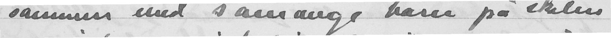sammen med så mange barn på skolen
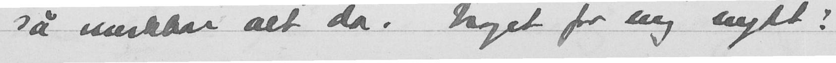så merkbar alt da. Noget for mig nytt:
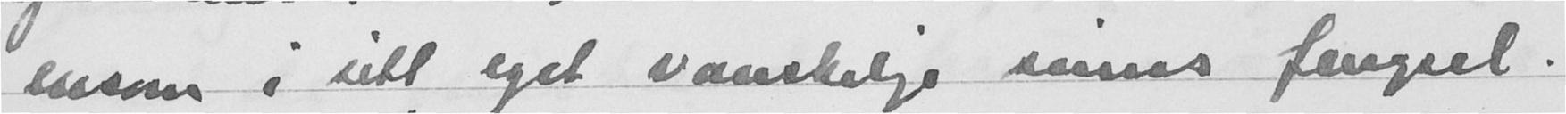ensom i sitt eget vanskelige sinns fengsel.
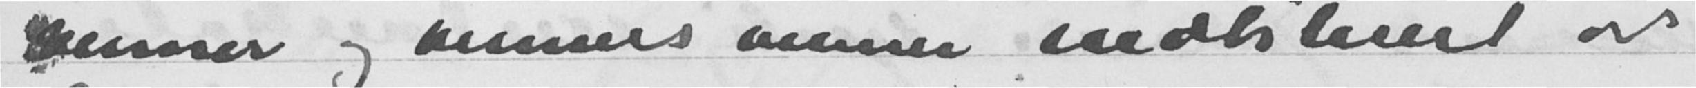venner og hennes uvenner mobilisert av
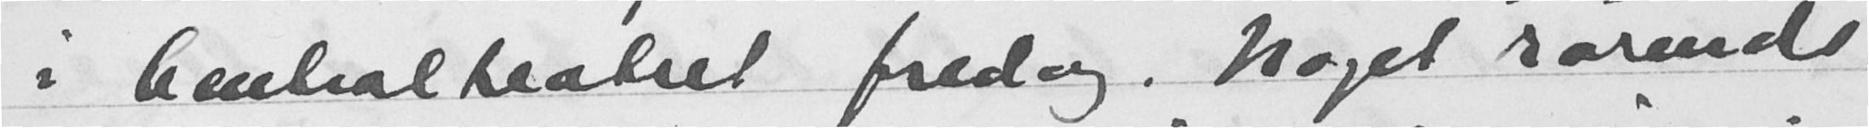i bentealteatret fredag. Noget rørende
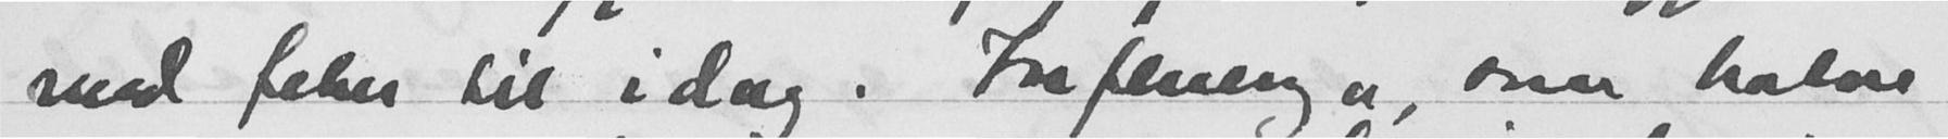med feber til i dag. Influenza, som halve
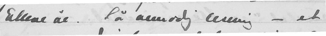Elleve år. Så vemodig lesning - et
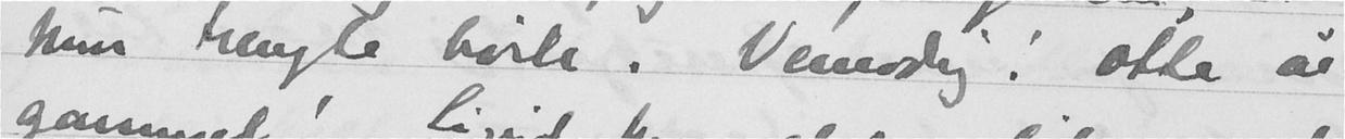hun trengte hvile. Vemodig! Åtte år
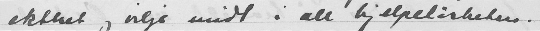ekthet og vilje midt i all hjelpeløsheten.
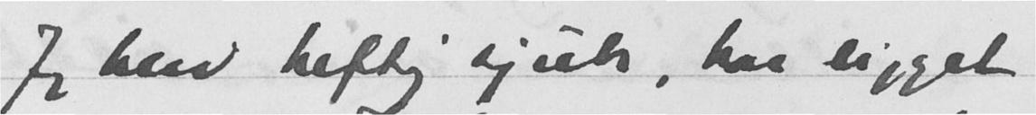Jeg blev heftig sjuk, har ligget
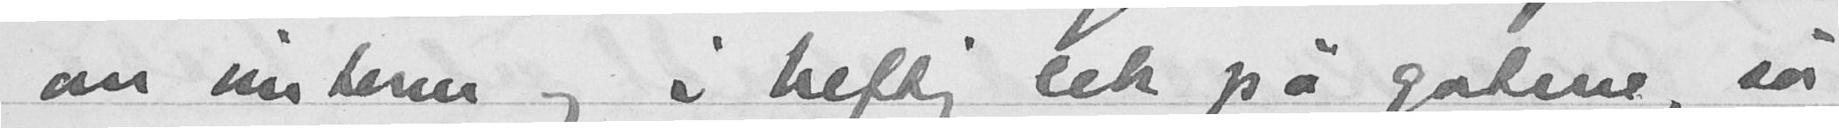om vinteren og i heftig lek på gatene, så
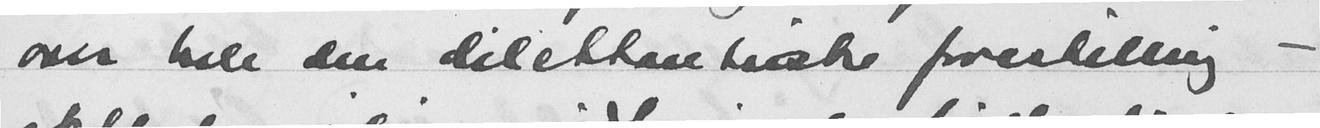over hele den dilettantiske foresteling -
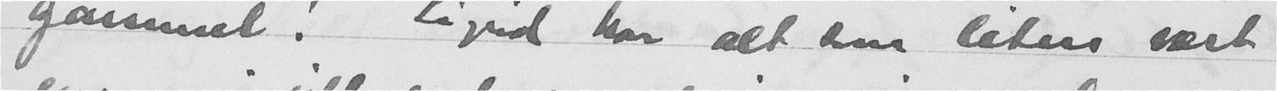gammel! Sigrid har alt som liten vært
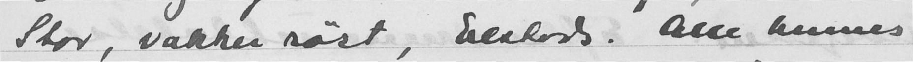Stor, vakker røst, Eestads. Alle hennes
